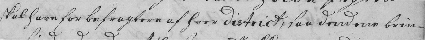skal have for befragtere af hver district, saa dend ene brin-
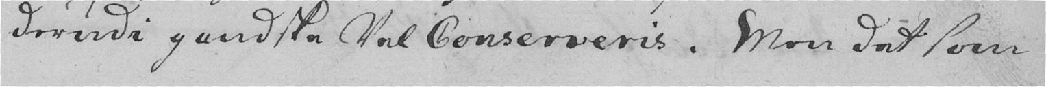derudi gandske Vel Conserveris. Men det som
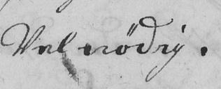Velnødig.
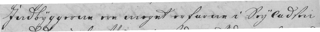Indbyggerne ere meget erfarne i Seyladser
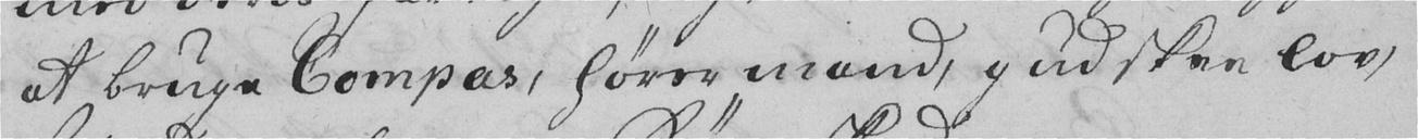at bruge Compas, hører mand, gud skee lov,
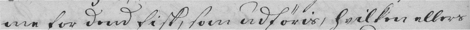me for dend fisk, som udføris, hvilken ellers
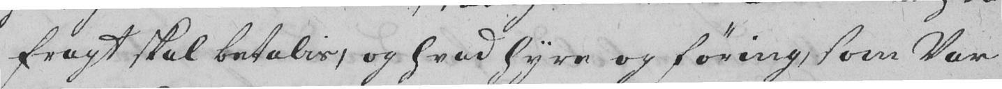fragt skal betalis, og hvad hyre og føring, som Var
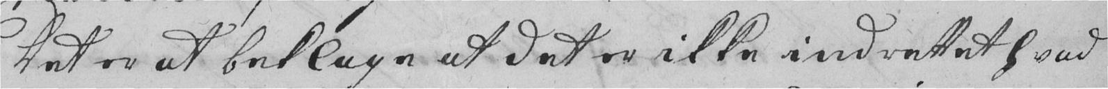Det er at beklage at det er ikke indrettet hvad
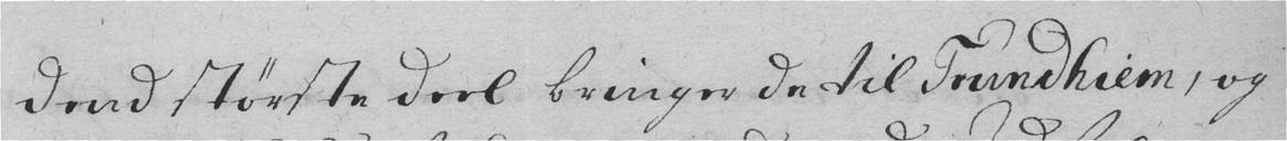dend største deel bringer de til Trundhiem, og
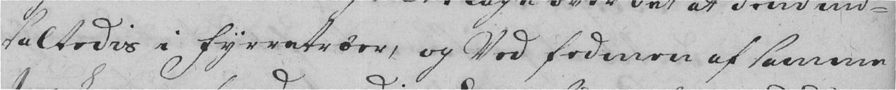saltedis i Fyrretræer, og Ved fodmen af samme
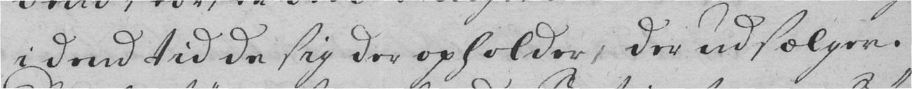i dend tid de sig der opholder, der udsælger.
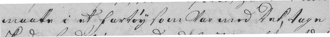maatte i et Fartøy som var med Dek, tage
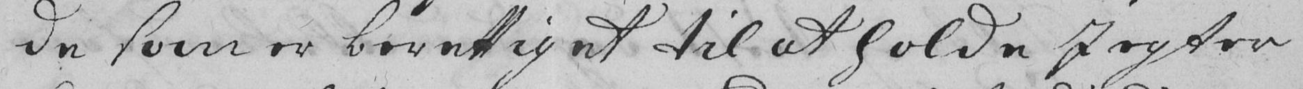de som er berettiget til at holde Iegter
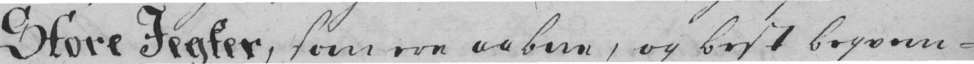Store Iegter, som ere aabne, og best beqvem-
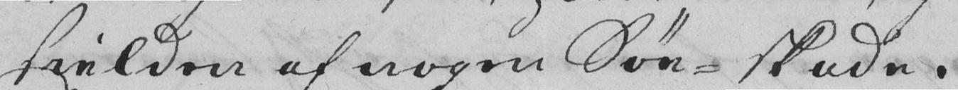sielden af nogen Søe-skade.
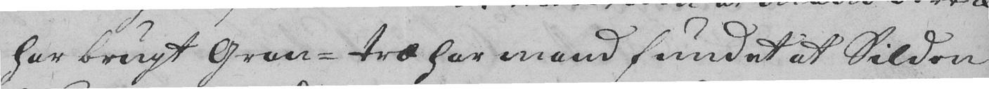har brugt Gran-træ har mand fundet at Silden
